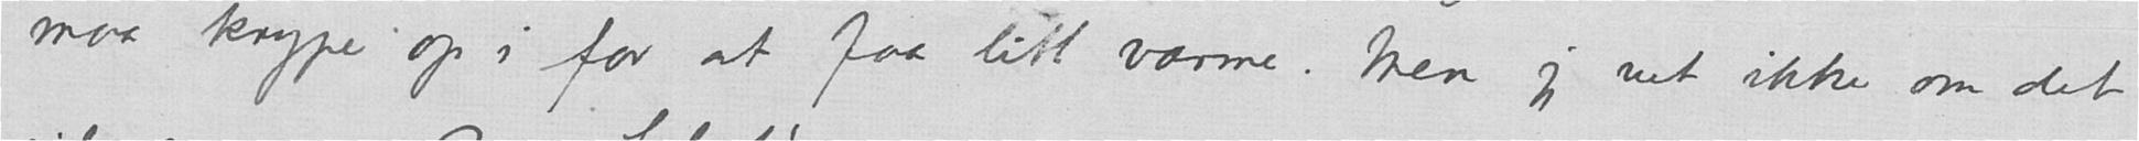maa krype op i for at faa litt varme. Men jeg vet ikke om det
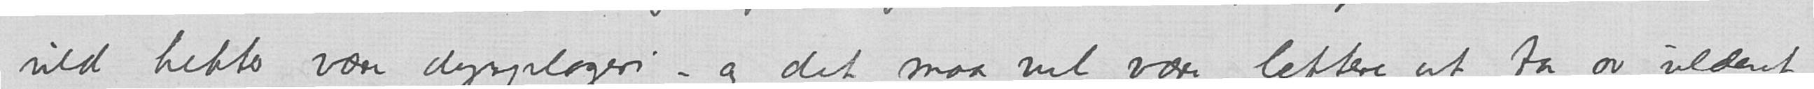uld hette være dyreplageri - og det maa vel være lettere at ta av uldent
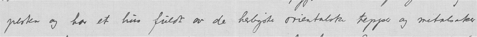pesten og har et hus fuldt av de herligste orientalske tepper og metalsaker
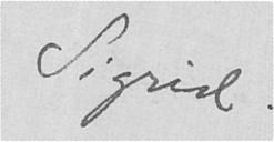Sigrid
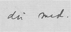du med.
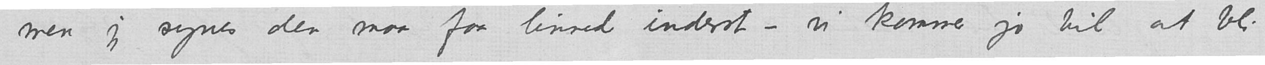men jeg synes den maa faa linned inderst — vi kommer jo til at bli
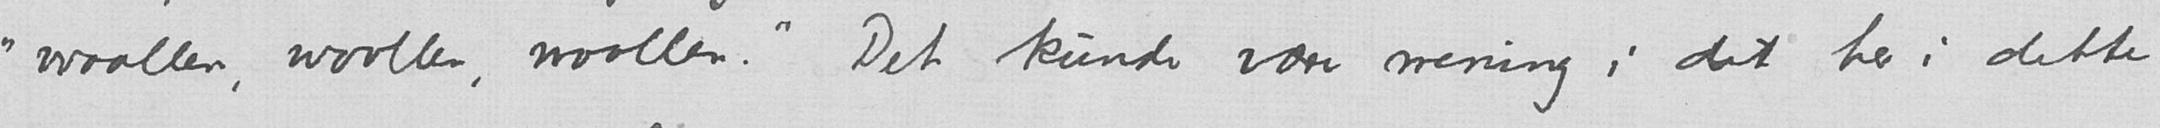«woollen, woollen, woollen». Det kunde være mening i det her i dette
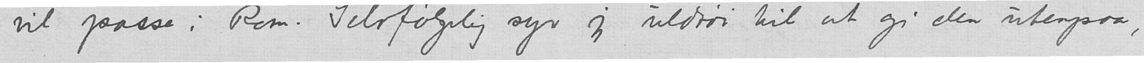vil passe i Rom. Selvfølgelig syr jeg uldtøi til at gi den utenpaa,
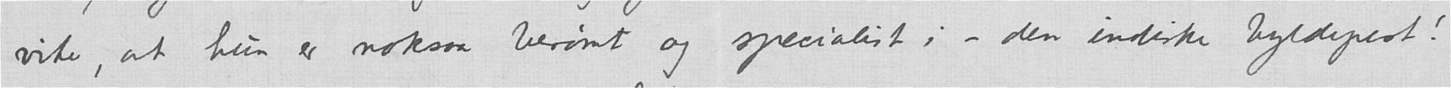vite, at hun er noksaa berømt og specialist i - den indiske byldepest!
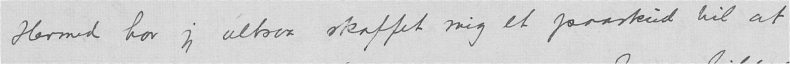Hermed har jeg altsaa skaffet mig et paaskud til at
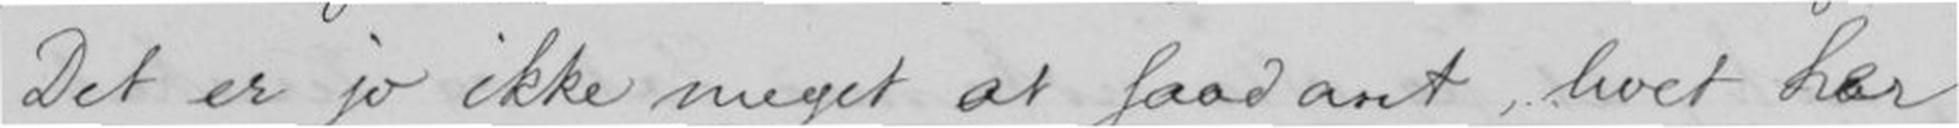Det er jo ikke meget at saadant livet her
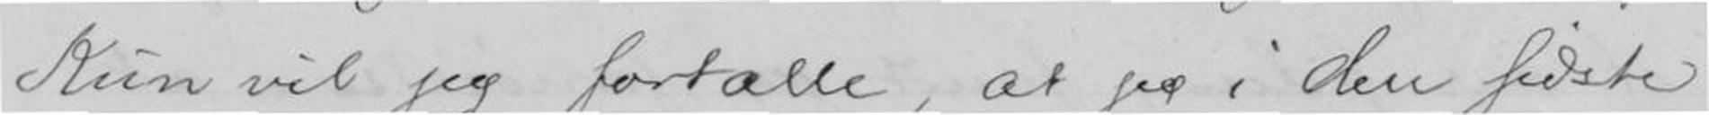Kun vil jeg fortælle, at jeg i den sidste
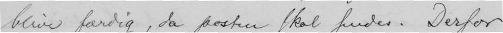blive færdig, da posten skal sendes. Derfor
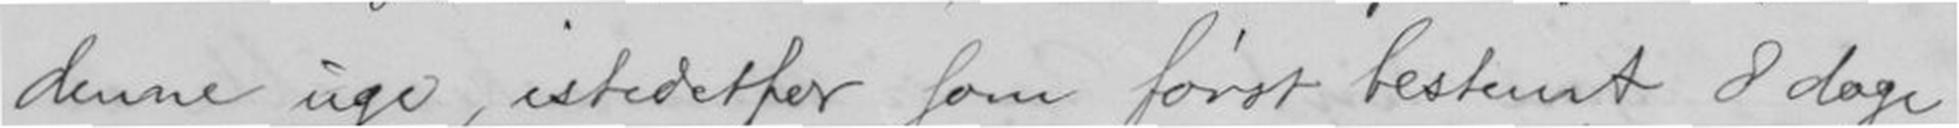denne uge, istedetfor som først bestemt 8 dage
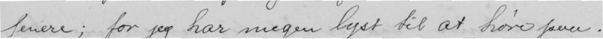senere; for jeg har megen lyst til at høre paa.
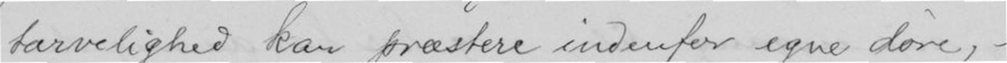tarvelighed kan præstere istenfor egne døre,
